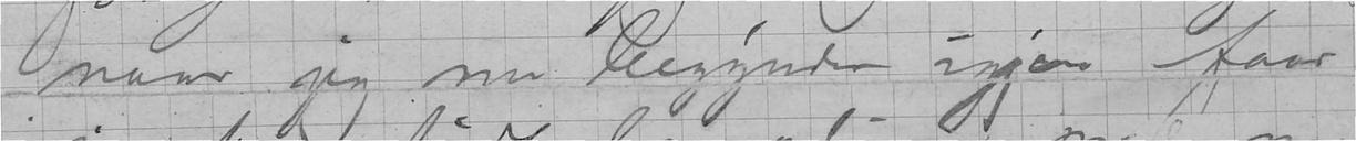naar jeg nu begynder igjen faar
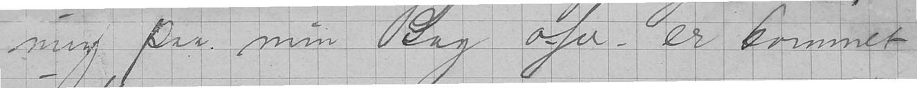ning paa min Bog o.s.v er kommet
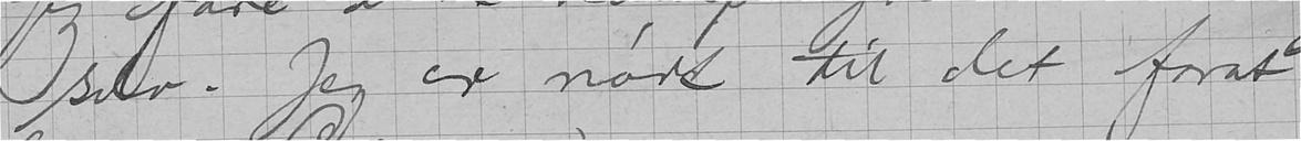selv. Jeg er nødt til det forat
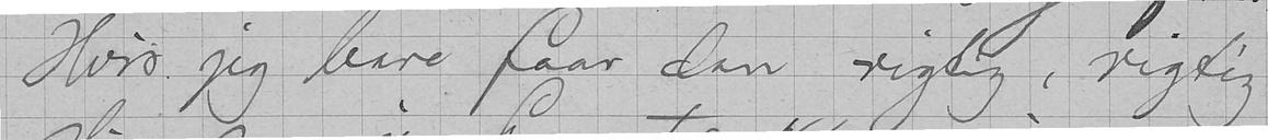Hvis jeg bare faar den rigtig, rigtig
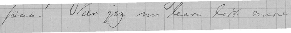paa! Var jeg nu bare lidt mere
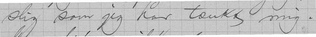slig som jeg har tænkt mig.
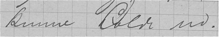kunne holde ud.
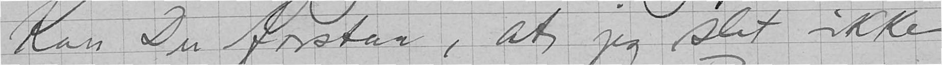Kan Du forstaa, at jeg slet ikke
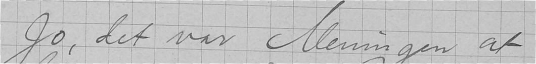Jo, det var Meningen at
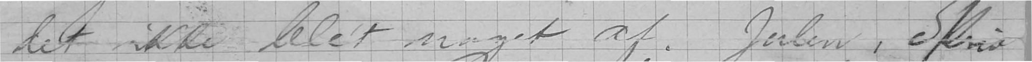det ikke blet noget af. Julen, Skriv-
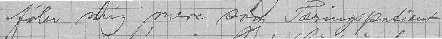føler mig mere som Tæringspatient
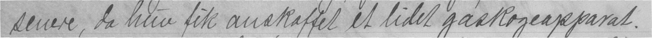senere, da hun fik anskaffet et lidet gaskogeapparat.
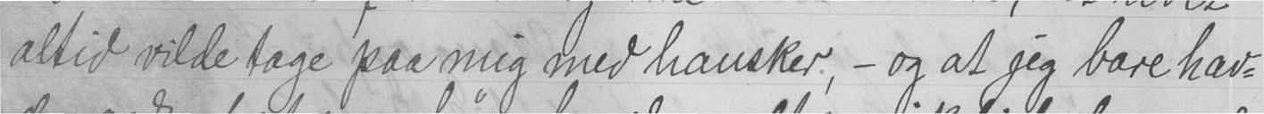altid vilde tage paa mig med hansker, - og at jeg bare hav-
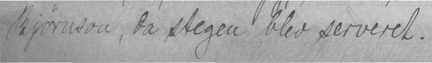Bjørnson, da stegen blev serveret.
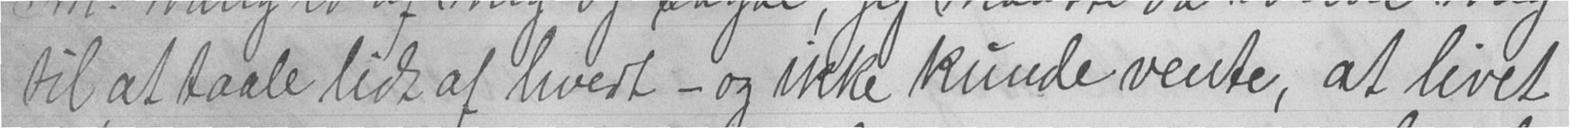til at taale lidt af hvert - og ikke kunde vente, at livet
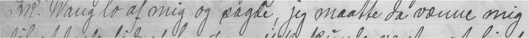Frk. Wang lo af mig og sagde, jeg maatte da vænne mig
